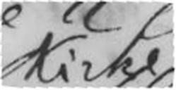Kirke
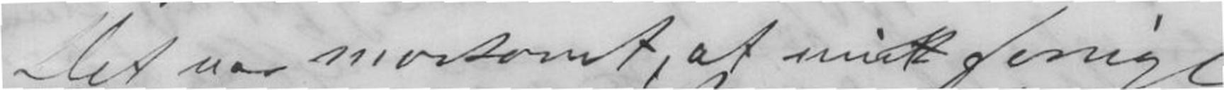Det var morsomt, at mit forrige
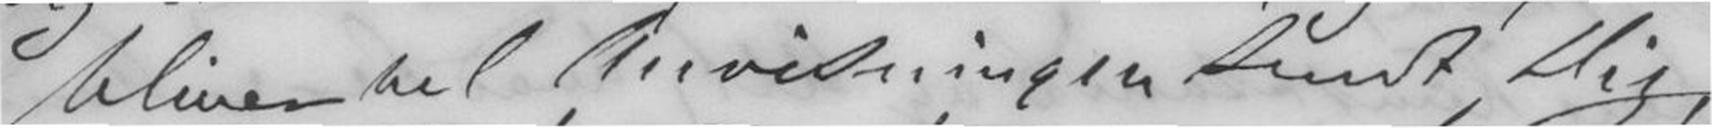bliver vel Anvisningen sendt Dig,
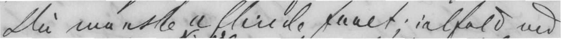Du maaske allerede faaet; ialfald ved
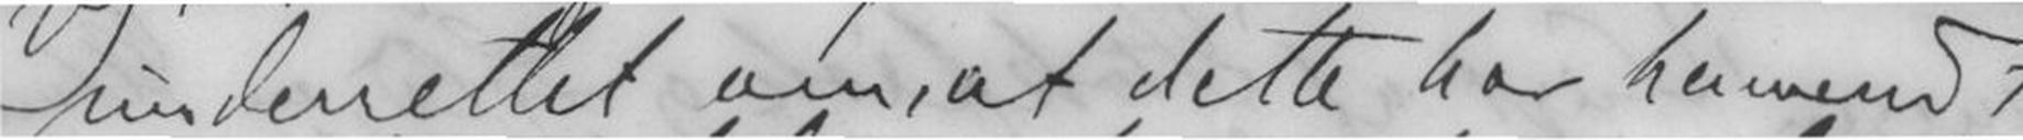underrettet om, at dette har henvendt
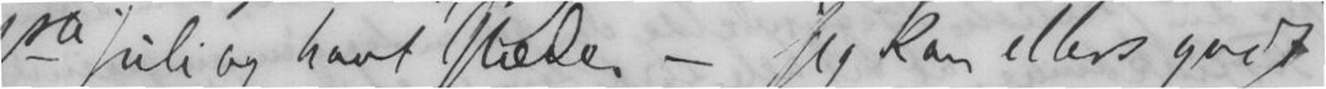1ste Juli og havt Glæder - Jeg kan ellers godt
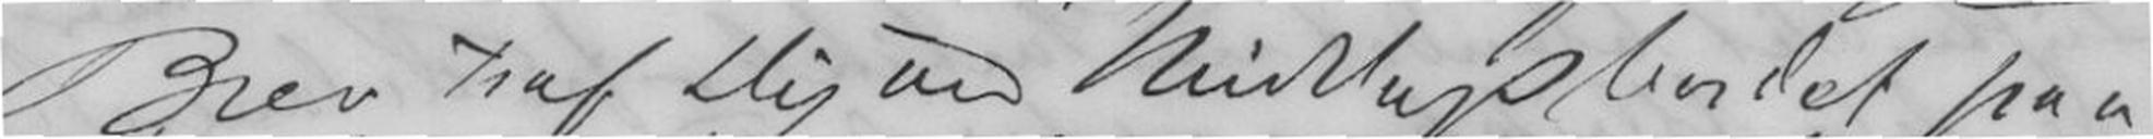Brev traf Dig ved Middagsbordet paa
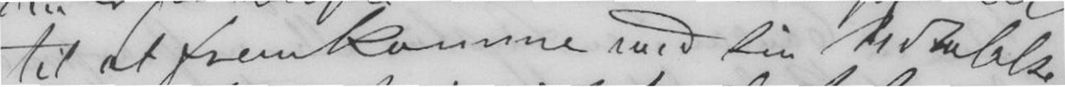til at fremkomme med sin Udtalelse:
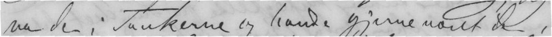var der i Tankerne og havde gjerne været der i
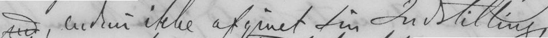ved, endnu ikke afgivet sin Indstilling,
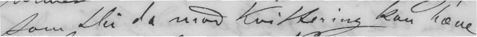som Du da mod Kvittering kan hæve
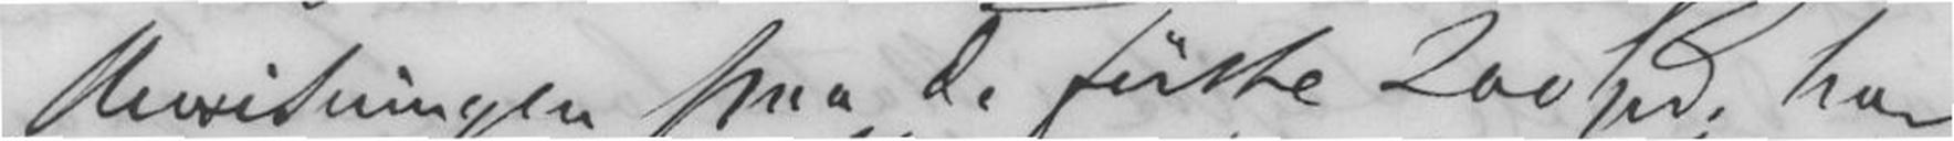Anvisningen paa de første 200 Spd. har
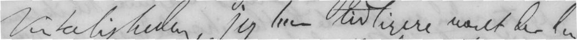Virkeligheden, jeg har tidligere været der den
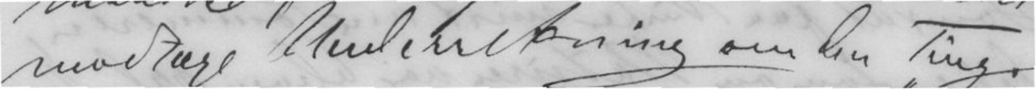modtage Underretning om den Ting.
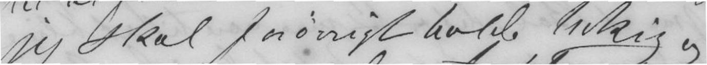jeg skal forøvrigt holde Udkig og,
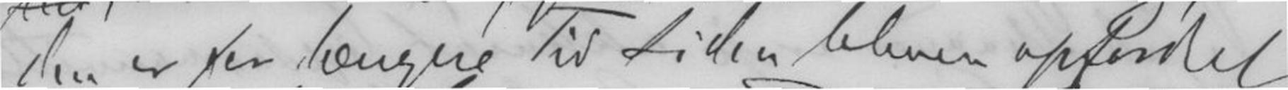den er for længere Tid siden bleven opfordret
